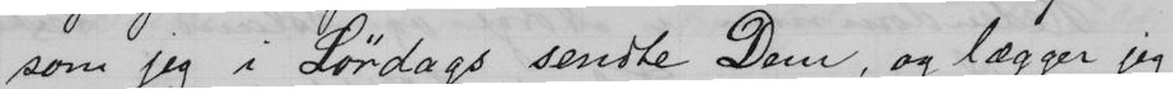som jeg i Lørdags sendte Dem, og lægger jeg
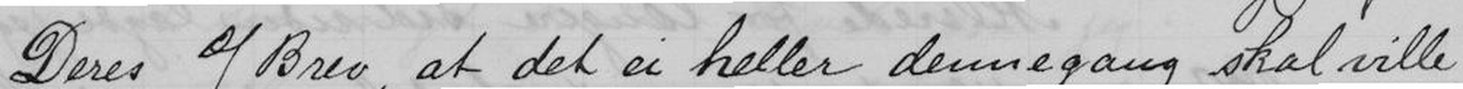Deres a/ Brev at det ei heller dennegang skal ville
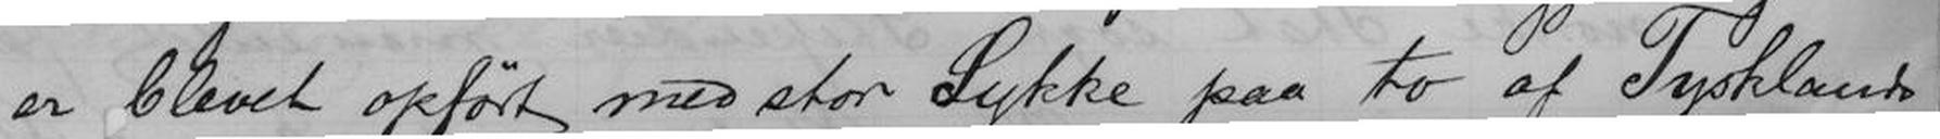er blevet opført med stor Lykke paa to af Tysklands
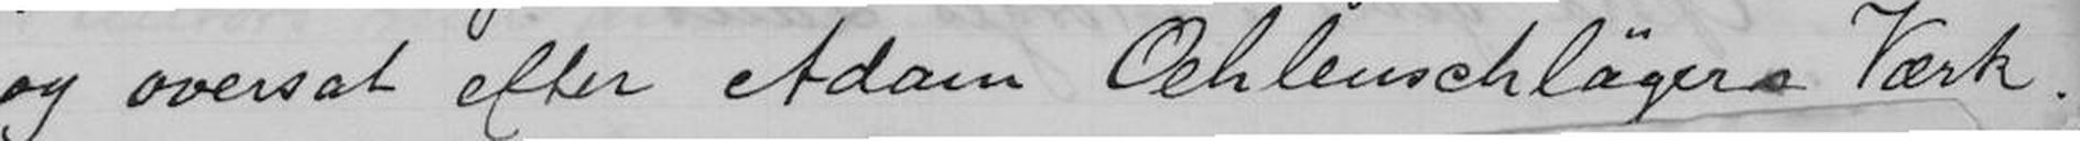og oversat efter Adam Oehlenschlägers Værk.
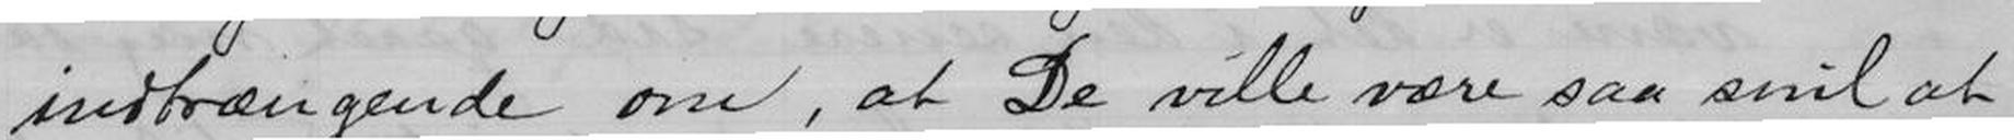indtrængende om, at De ville være saa snil at
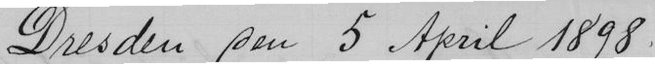Dresden den 5 April 1898.
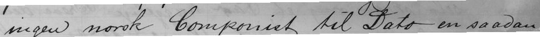ingen norsk Componist til Dato en saadan
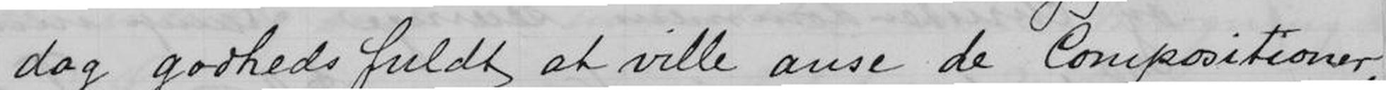dog godhedsfuldt at anse de Compositioner,
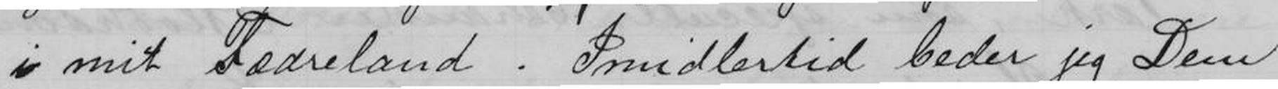i mit Fædreland. Imidlertid beder jeg Dem
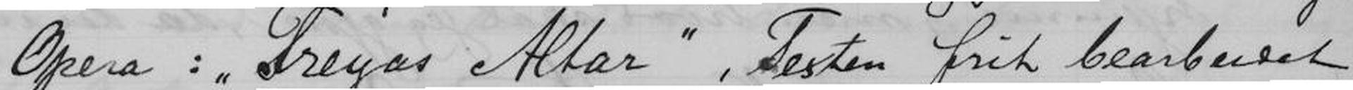Opera: "Freyas Altar". Texten frit bearbeidet
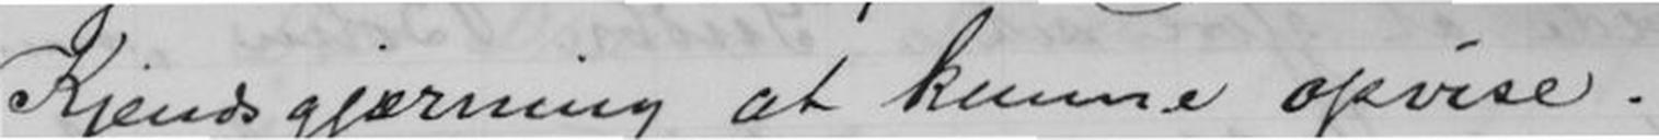Kjendsgjærning at kunne opvise. -
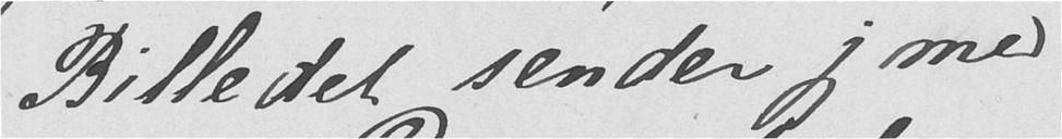Billedet sender jeg med
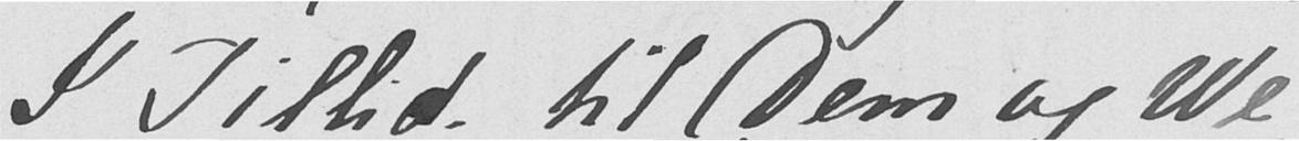I Tillid til Dem og We-
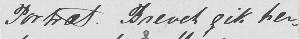Portræt. Brevet gik her-
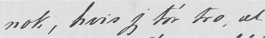nok, hvis jeg tør tro, at
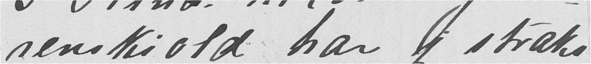renskiold har jeg straks
